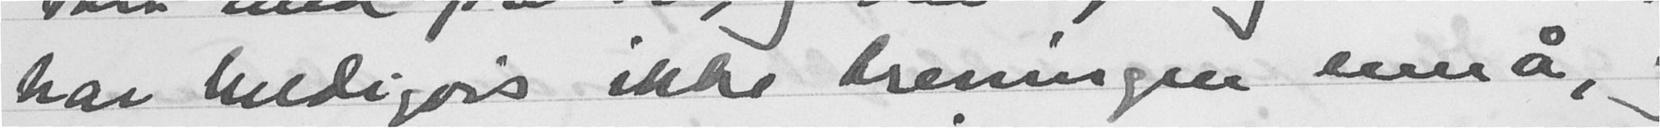har heldigvis ikke treningen ennå, og
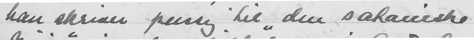han skriver pussig til "den sataniske
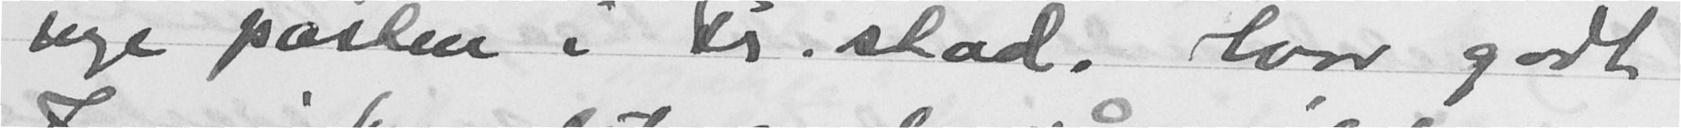nye posten i Fr. stad. Hvor godt
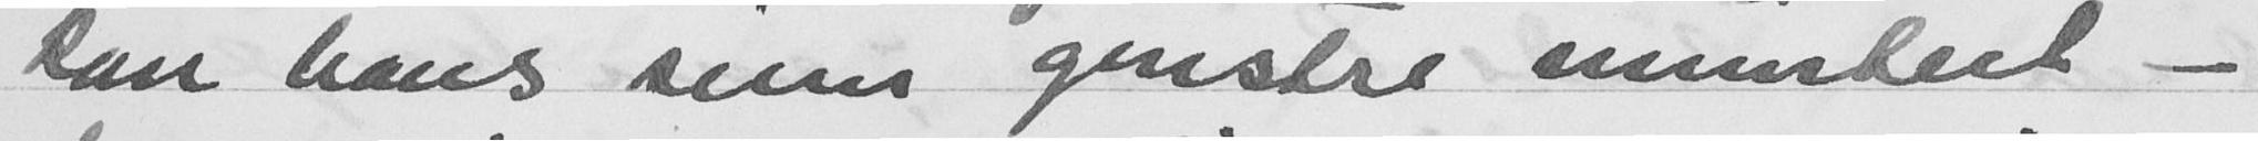kan hans sinn gnistre muntert -
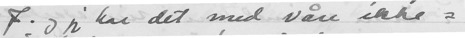J. og jeg har det med våre ikke-
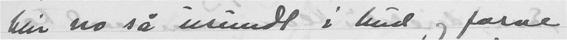blir no så usundt i hud og farve
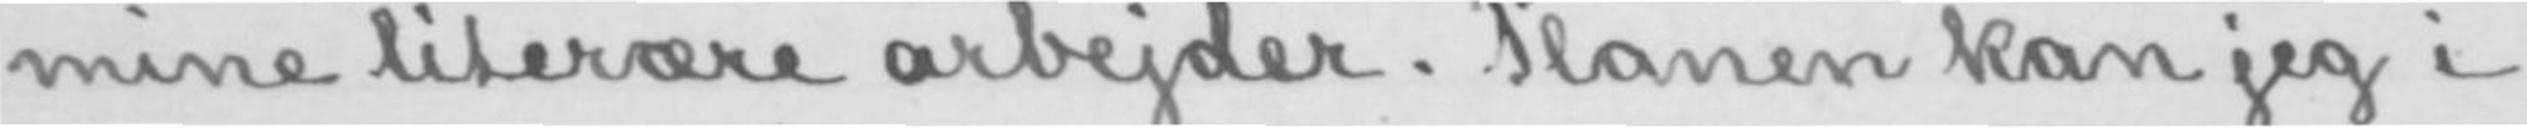mine literære arbejder. Planen kan jeg i
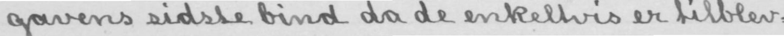gavens sidste bind da de enkeltvis er tilblev-
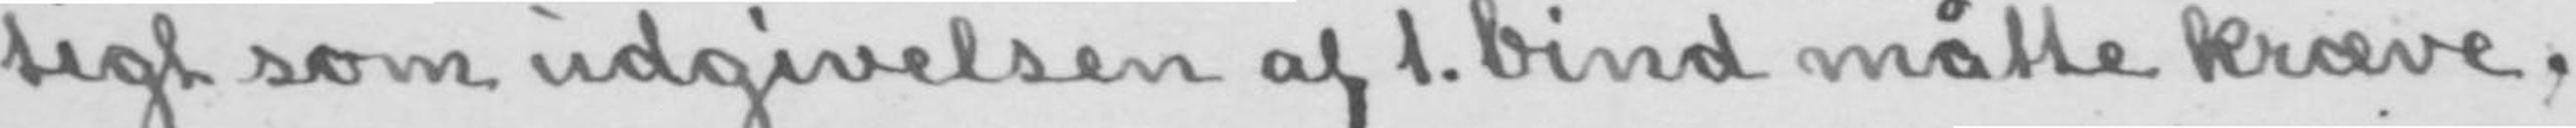tigt som udgivelsen af 1. bind måtte kræve.
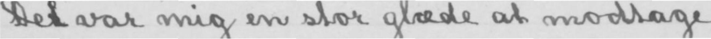Dert var mig en stor glæde at modtage
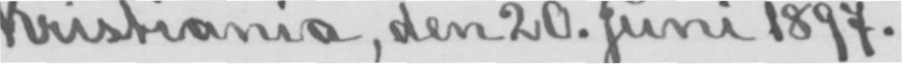Kristiania, den 20. Juni 1897.
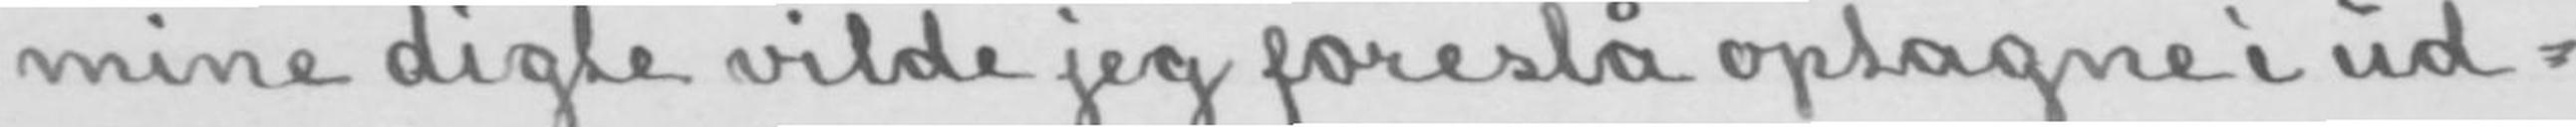mine digte vilde jeg foreslå optagne i ud-
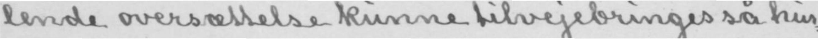lende oversættelse kunne tilvejebringes så hur-
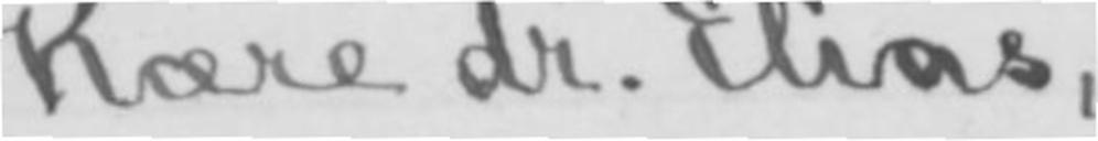Kære dr. Elias,
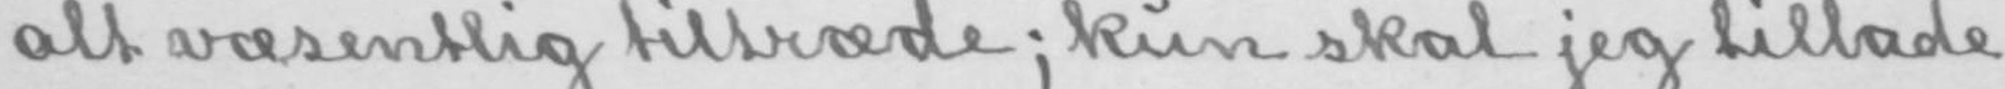alt væsentlig tiltræde; kun skal jeg tillade
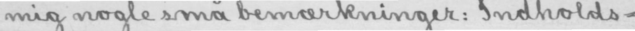mig nogle små bemærkninger: Indholds-
